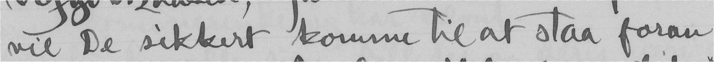vil De sikkert komme til at staa foran
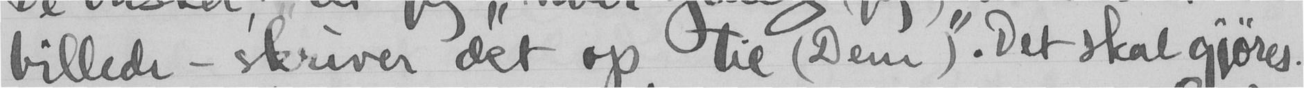billede - skriver det op til (Dem)". Det skal gjøres.
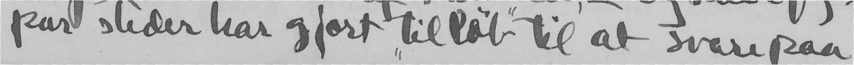par steder har gjort "tilløb" til at svare paa
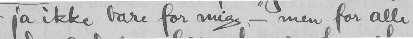ja ikke bare for mig, - men for alle
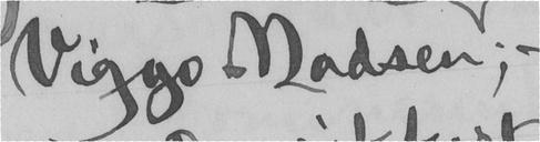Viggo Madsen; -
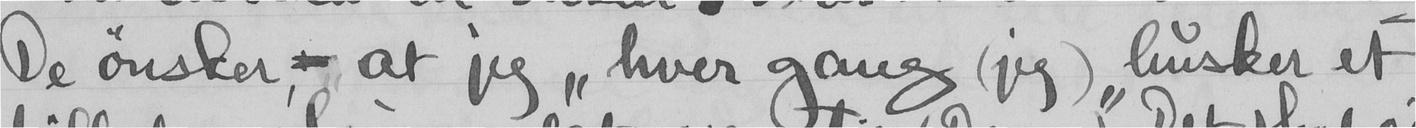De ønsker - at jeg "hver gang (jeg) husker et
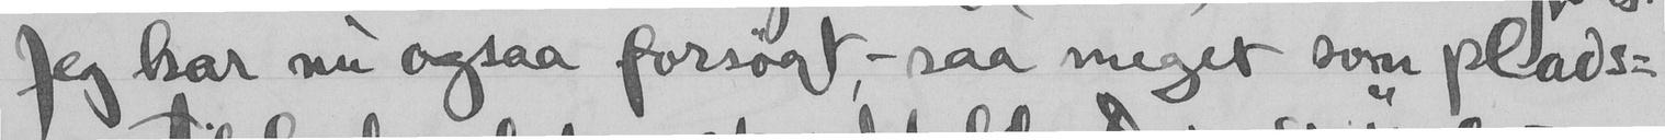Jeg har nu ogsaa forsøgt, - saa meget som plads-
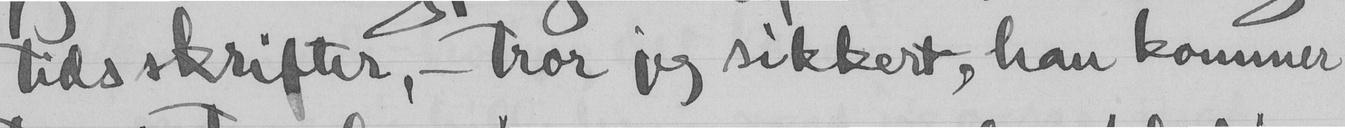tidsskrifter, - tror jeg sikkert, han kommer
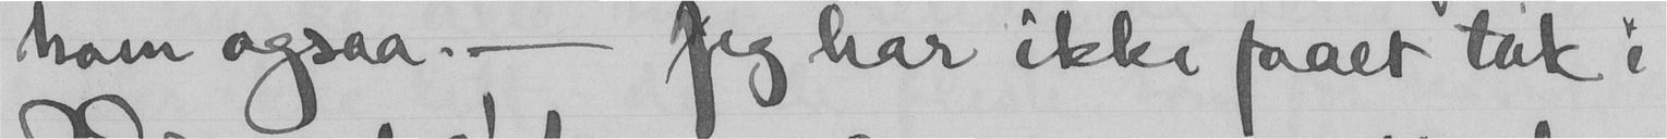ham ogsaa. - Jeg har ikke faaet tak i
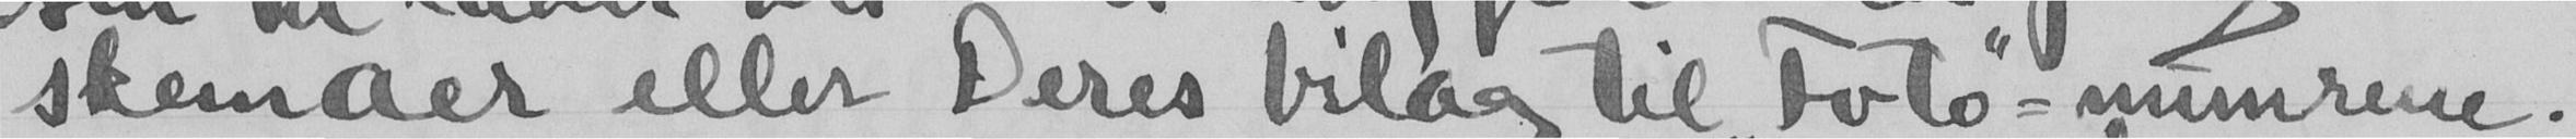skemaer eller Deres bilag til "Foto"-numrene.
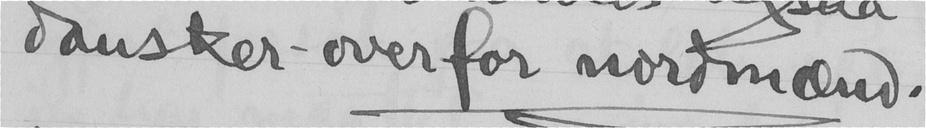dansker - overfor nordmænd.
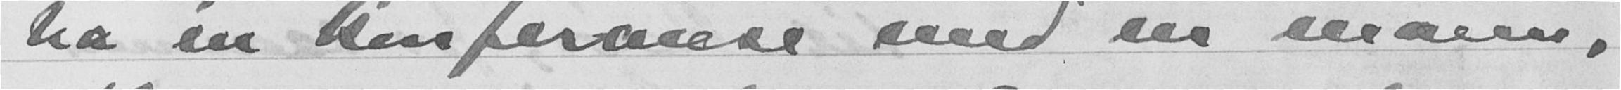ha en konferanse med en mann,
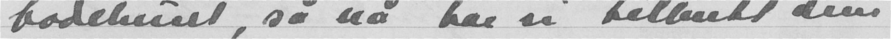badehuset, så nå har vi tilbudt dem
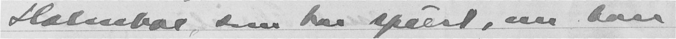Holmboe, som har spurt, om han
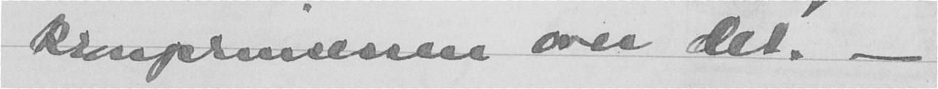kronprinsessen over det. -
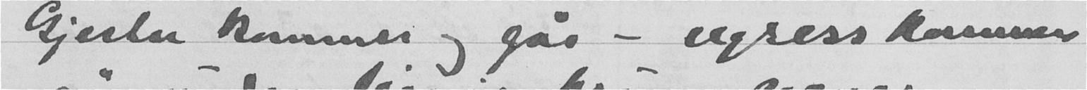Gjester kommer og går - ugress kommer
